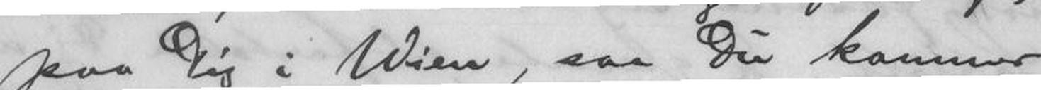paa Dig i Wien, saa Du kommer
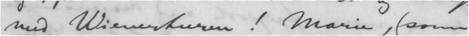med Wienerturen! Marie, (som
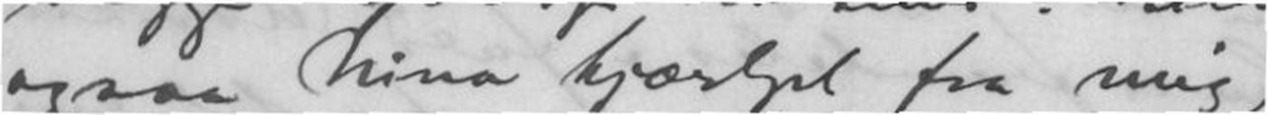ogsaa Nina kjærligst fra mig,
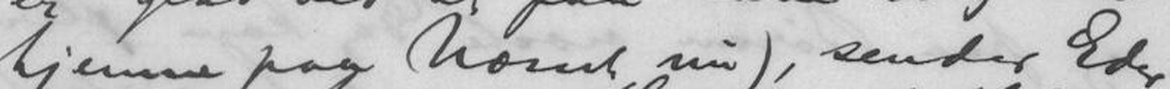hjemme paa Næsset nu), sender Eder
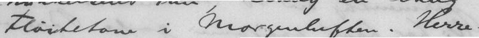Fløitetone i Morgenluften. Herre-
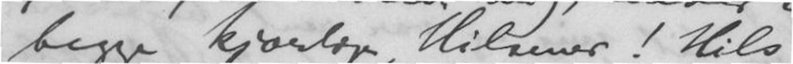begge kjærlige Hilsener! Hils
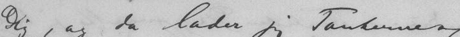Dig, og da lader jeg Tankerne og
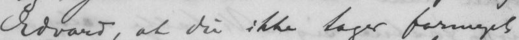Edvard, at du ikke tager formeget
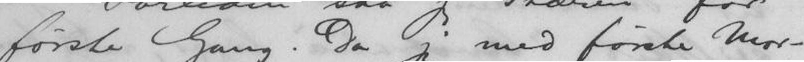første Gang. Da jeg med første Mor-
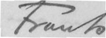Frants.
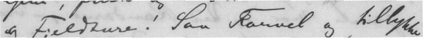og Fjeldture! Saa Farvel og tillykke
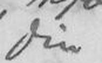Din
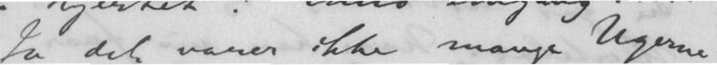Ja det varer ikke mange Ugerne
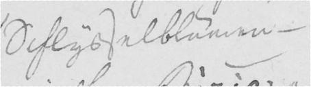Siflyselblumen -
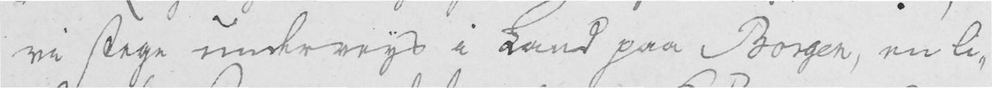vi stege underveys i Land paa Borgen, en li-
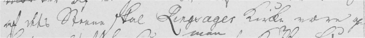af dets Steene skal Ringsager Kirke være op-
